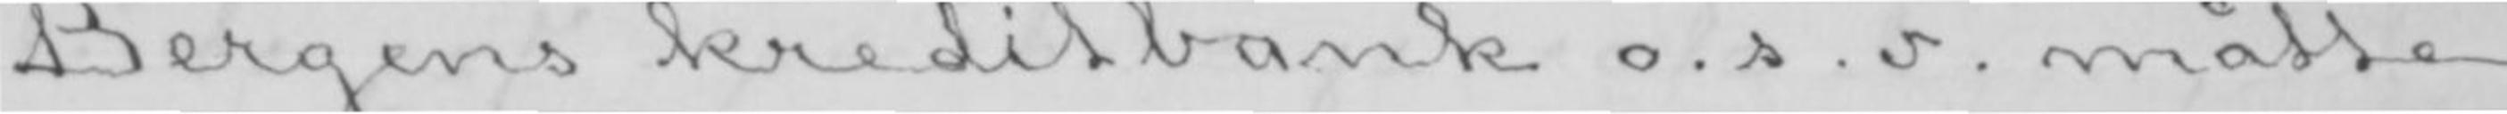Bergens kreditbank o. s. v. måtte
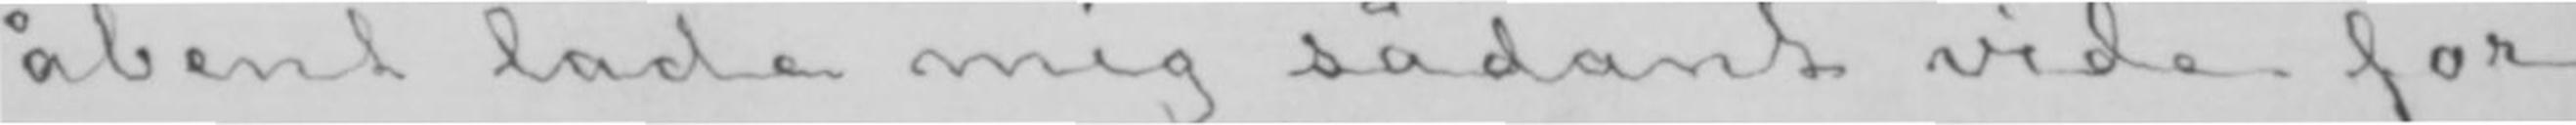åbent lade mig sådant vide for
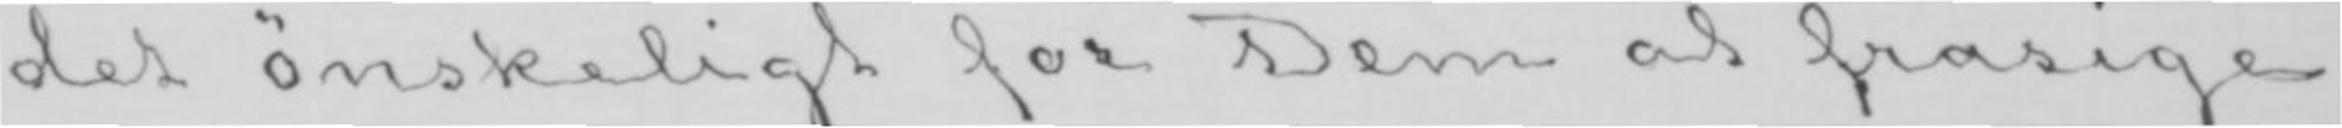det ønskeligt for Dem at frasige
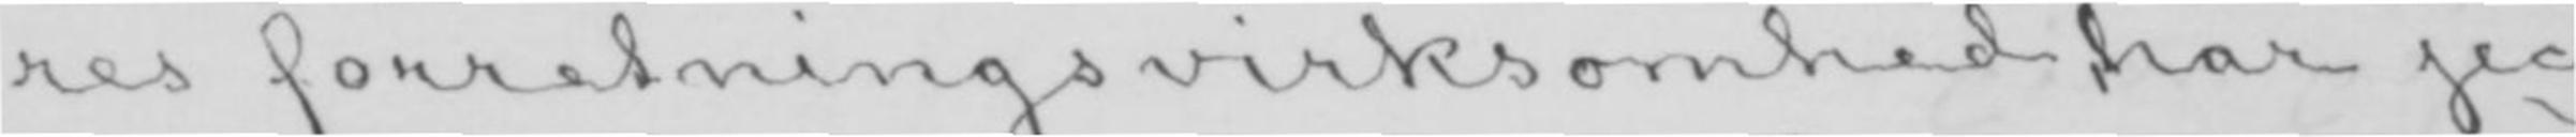res forretningsvirksomhed, har jeg
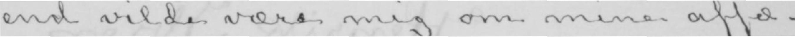end vilde være mig om mine affæ-
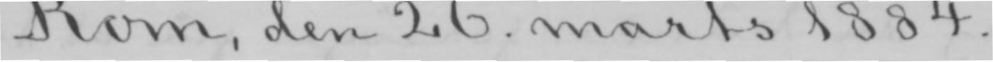Rom, den 26. marts 1884.
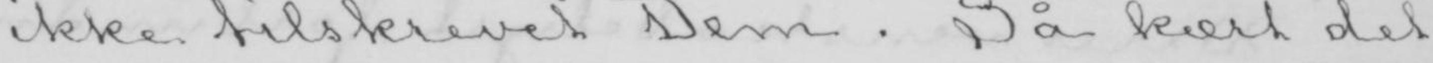ikke tilskrevet Dem. Så kært det
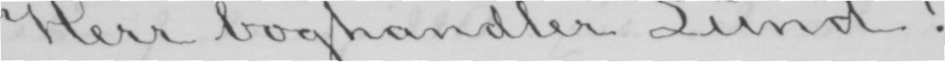Herr boghandler Lund!
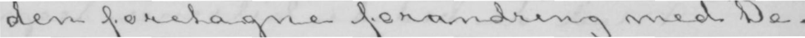den foretagne forandring med De-
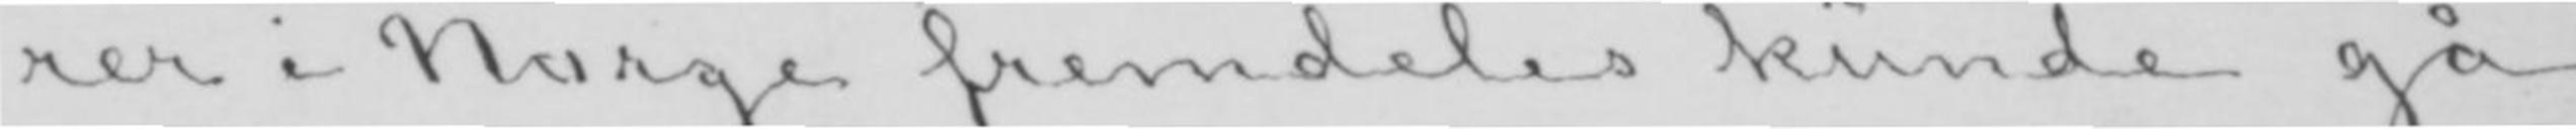rer i Norge fremdeles kunde gå
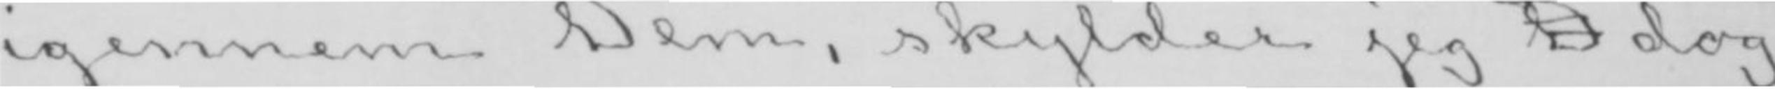igennem Dem, skylder jeg D dog,
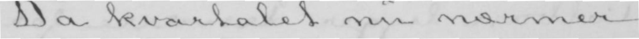Da kvartalet nu nærmer
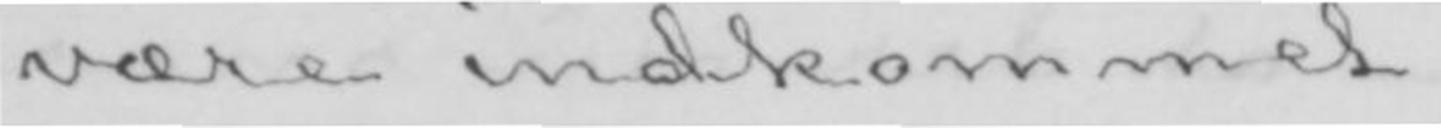være indkommet.
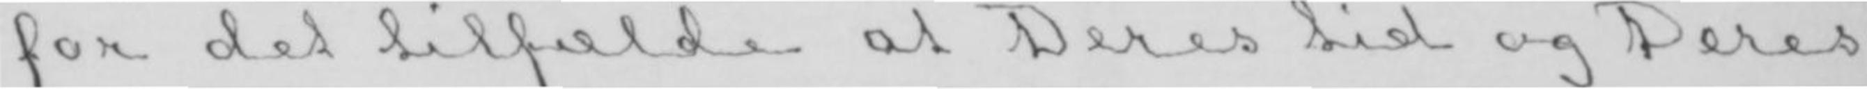for det tilfælde at Deres tid og Deres
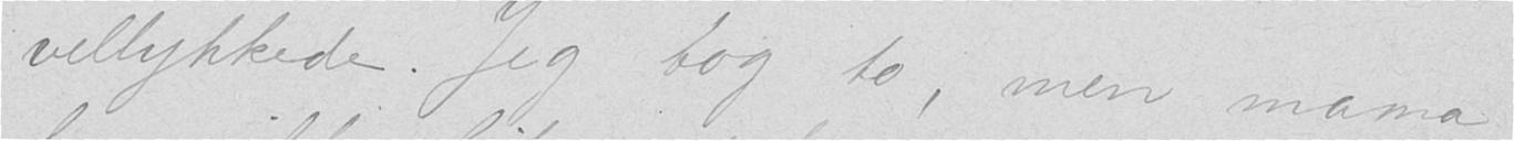vellykkede. Jeg tog to, men mama
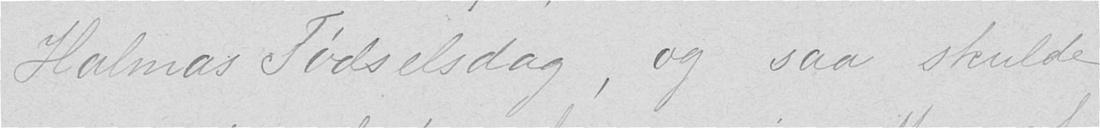Halmas Fødselsdag, og saa skulde
